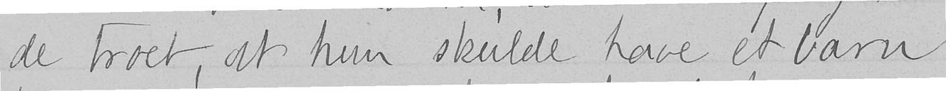de troet, at hun skulde have et barn
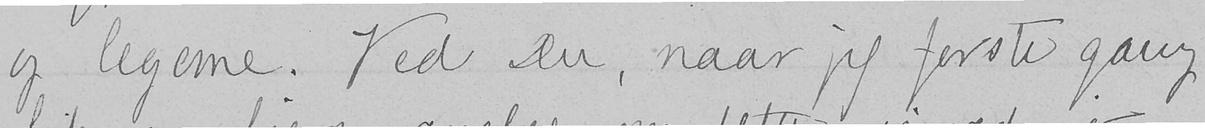og legeme. Ved Du, naar jeg første gang
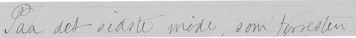Paa det sidste møde, som forresten
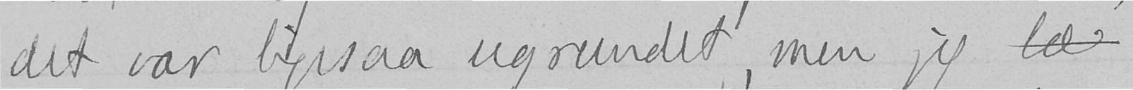det var ligesaa ugrundet, men jeg læ
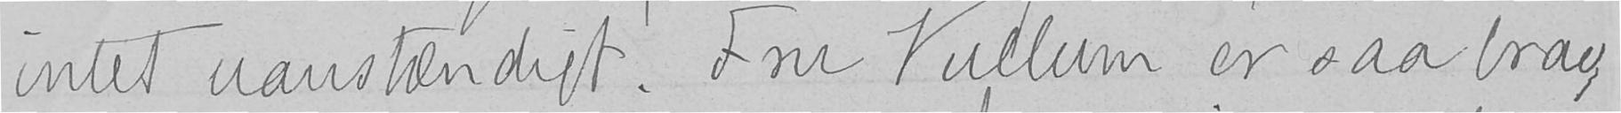intet uanstændigt. Fru Vullum er saa brav,
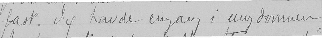fast. Jeg havde engang i ungdommen
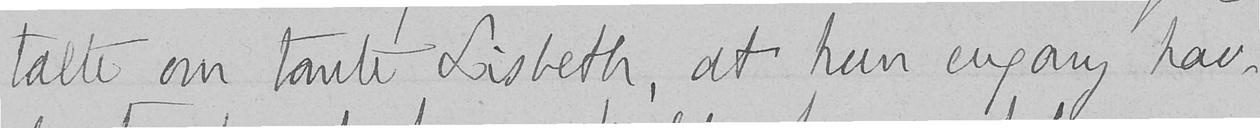talte om tante Lisbeth, at hun engang hav-
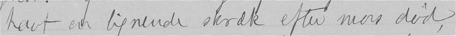havt en lignende skræk efter mors død
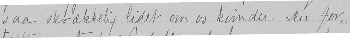saa skrækkelig lidet om os kvinder. Du for-
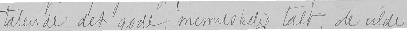talende det gode, menneskelig talt, de vilde
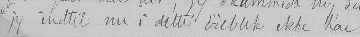at jeg indtil nu i dette øieblik ikke har
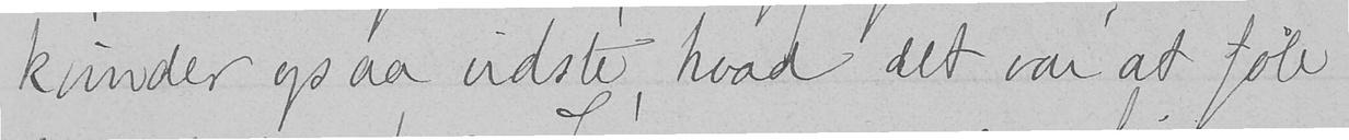kvinder ogsaa vidste, hvad det var at føle
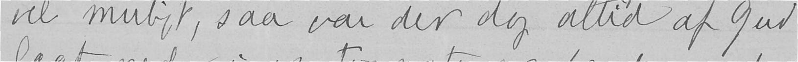vel muligt, saa var der dog altid af Gud
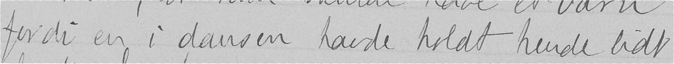fordi en i dansen havde holdt hende lidt
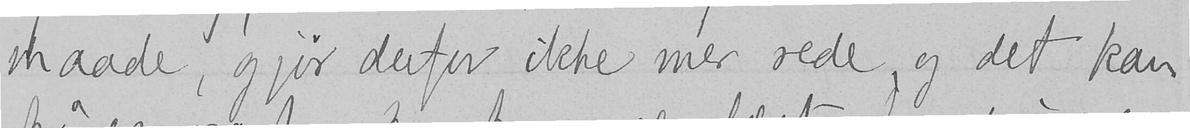maade, gjør derfor ikke mer rede, og det kan
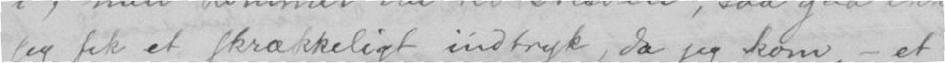Jeg fik et skrækkeligt indtryk, da jeg kom, - et
